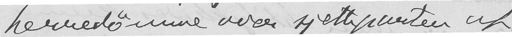herredømme over sjetteparten af
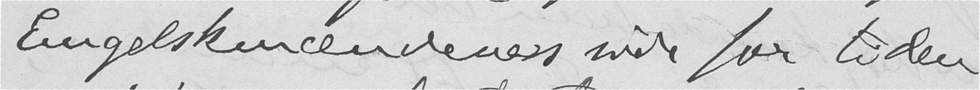Engelskmændenes side for tiden,
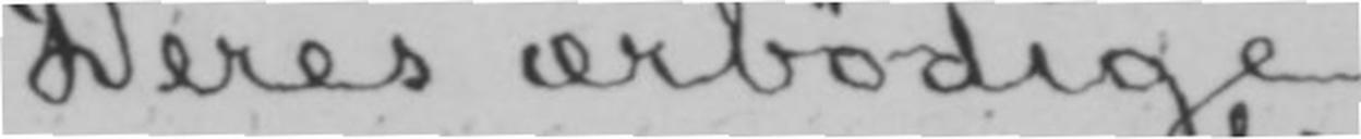Deres ærbødige
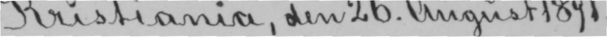Kristiania, den 26. August 1891.
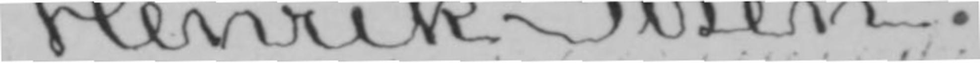Henrik Ibsen.
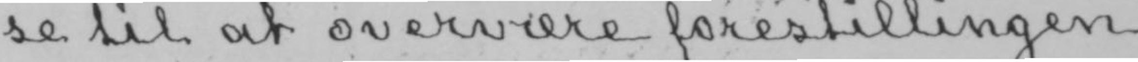se til at overvære forestillingen
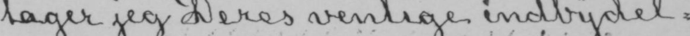tager jeg Deres venlige indbydel-
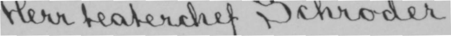Herr teaterchef Schrøder,
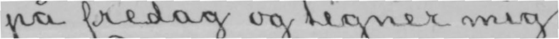på fredag og tegner mig
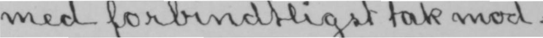med forbindtligst tak mod-
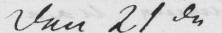Den 21de
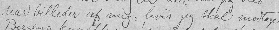har billeder af mig, hvis jeg skal modtage
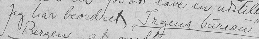Jeg har beordret "Irgens bureau"
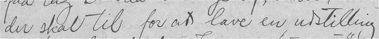der skal til for at lave en udstilling
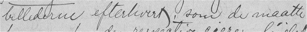billederne efterhvert, som de maatte
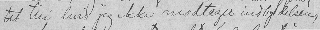thi hvis jeg ikke modtager indbydelsen,
